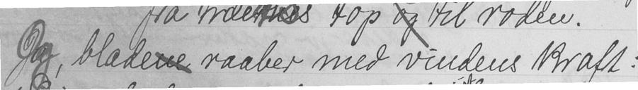Og, bladene raaber med vindens kraft:
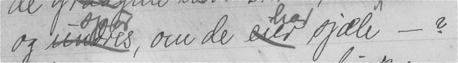og spår, om de har sjæle - ?
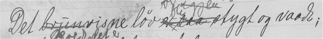Det brunvisne løv ble stygt og vaadt;
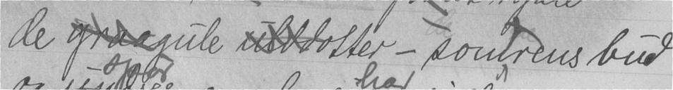de graagule ulddotter - somrens bud
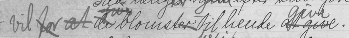- vil blomster til hende give.
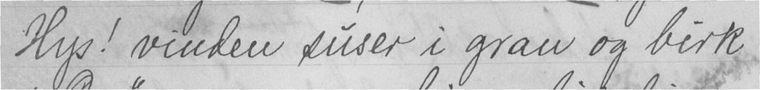Hys! vinden suser i gran og birk:
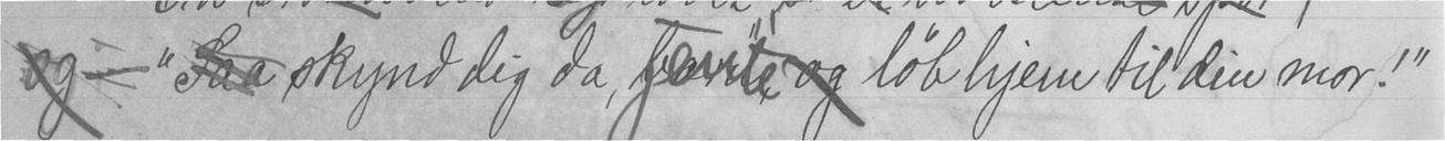- "skynd dig da, jente, løb hjem til din mor!"
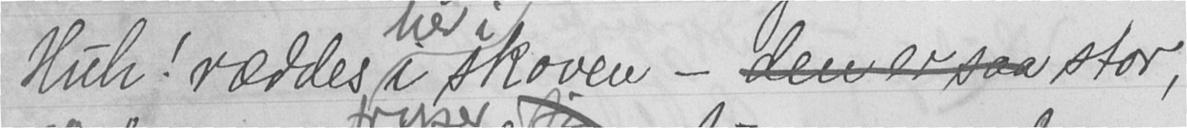Huh! ræddes her i skoven - den er saa stor,
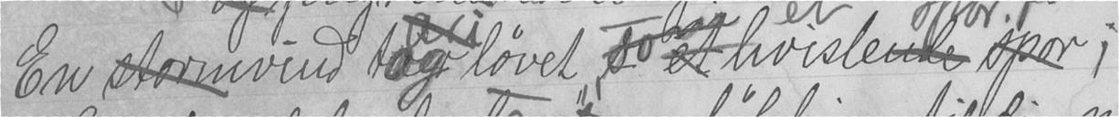En stormvind tag løvet, et hvislende spor;
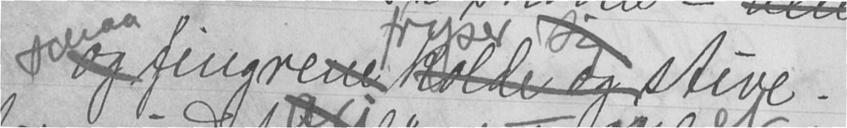smaa fingrene fryser stive.
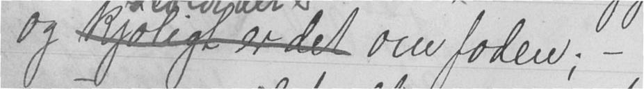og koldt det er om foden; -
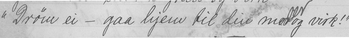"Drøm ei - gaa hjem til din moder virk!"
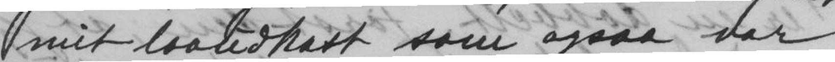mit lovudkast som ogsaa var
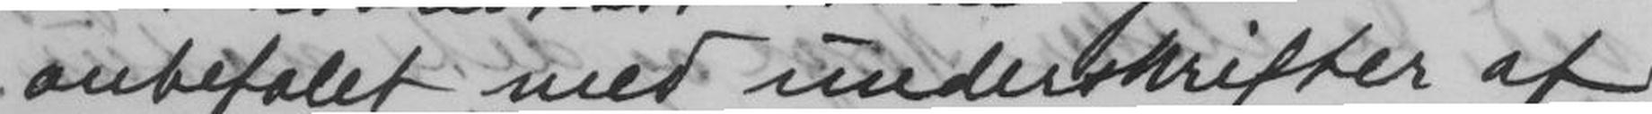anbefalet med underskrifter af
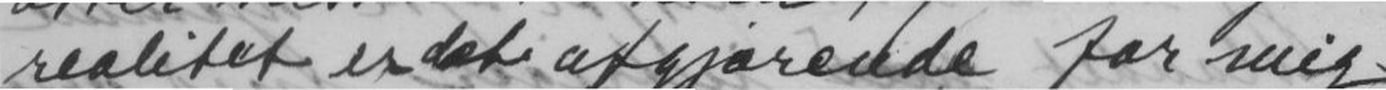realitet er det afgjørende for mig
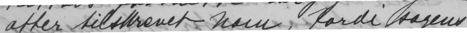atter tilskrevet ham, fordi sagens
